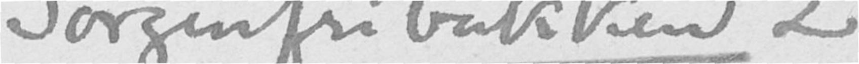Sorgenfribakken 2
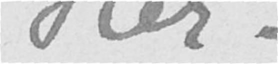Her.
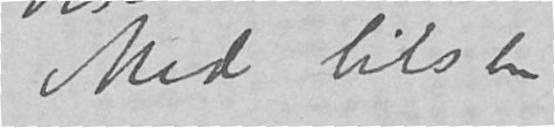Med hilsen
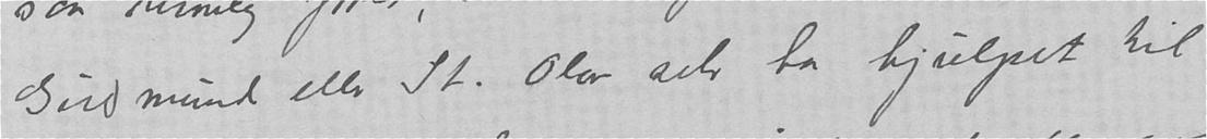Gudsmund eller St. Olav selv ha hjulpet til -
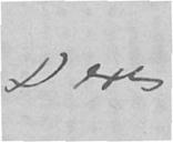Deres
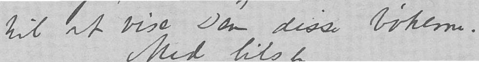til at vise Dem disse bøkerne.
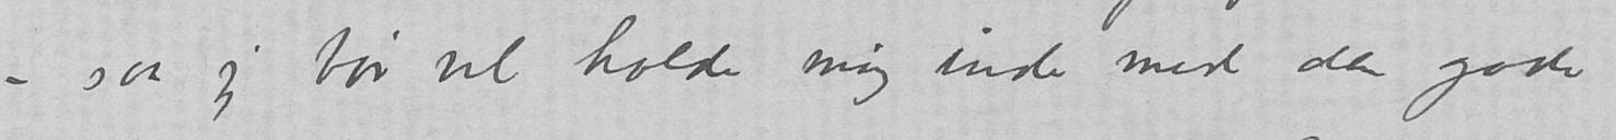saa jeg bør vel holde mig inde med den gode
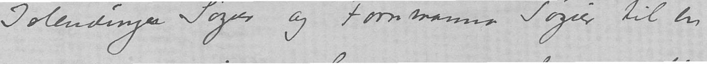Islendinga Sögur og Fornmanna Sögur til en
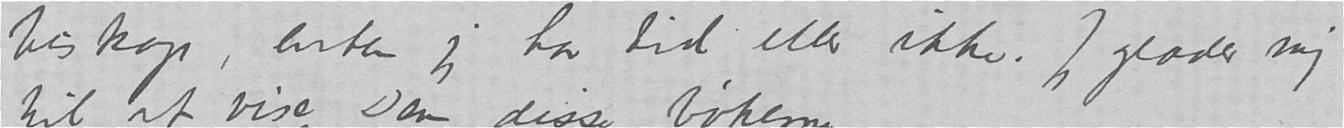biskop, enten jeg har tid eller ikke. Jeg glæder mig
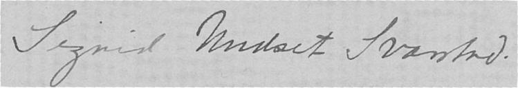Sigrid Undset Svarstad.
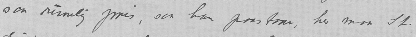saa rimelig pris, saa han paastaar, her maa St.
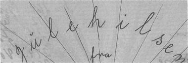julehilsen
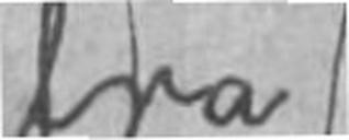fra
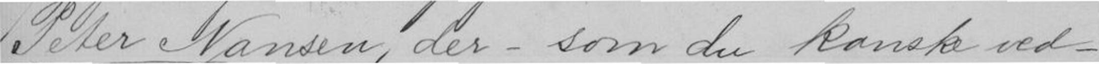Peter Nansen, der - som du kanske ved -
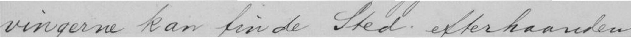vingerne kan finde Sted efter haanden
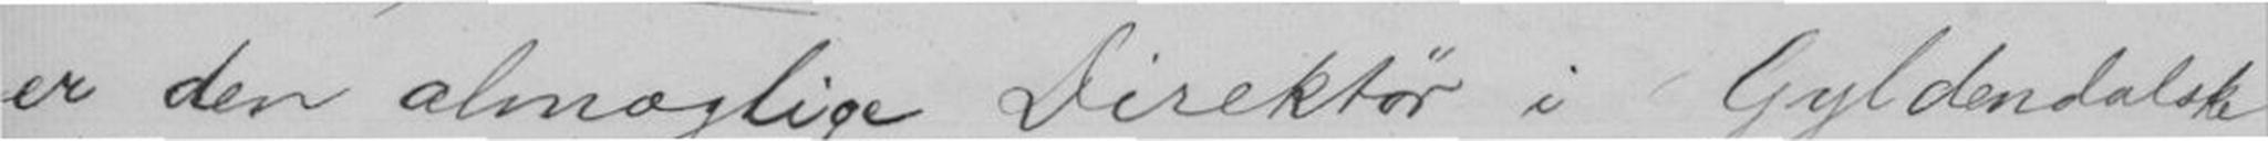er den almægtige Direktør i Gyldendalske
